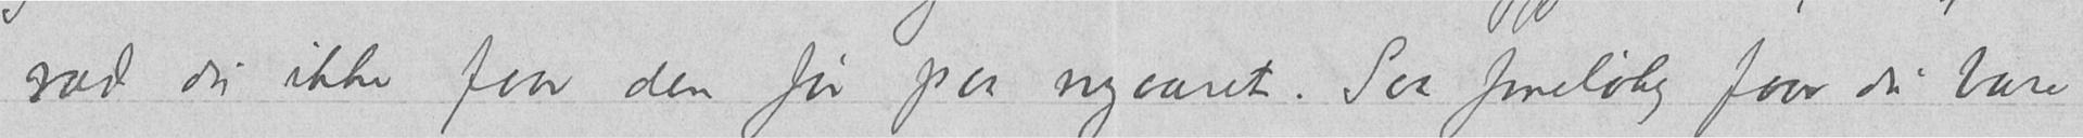ræd du ikke faar den før paa nyaaret. Saa foreløbig faar du bare
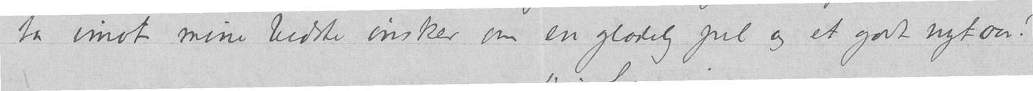ta imot mine bedste ønsker om en glædelig jul og et godt nytaar!
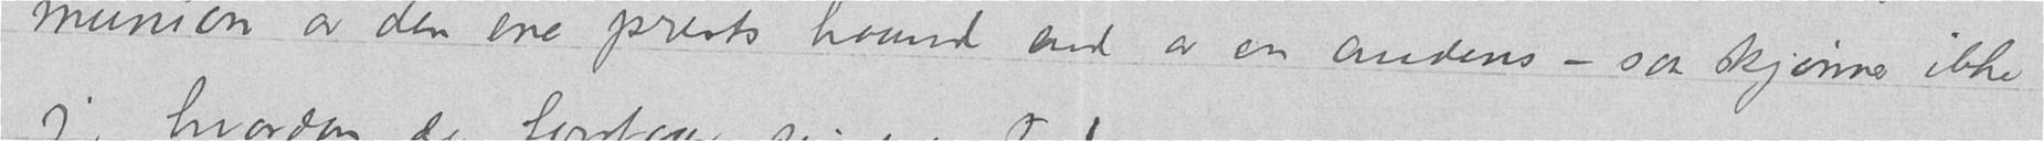munion av den ene prests haand end av en andens – saa skjønner ikke
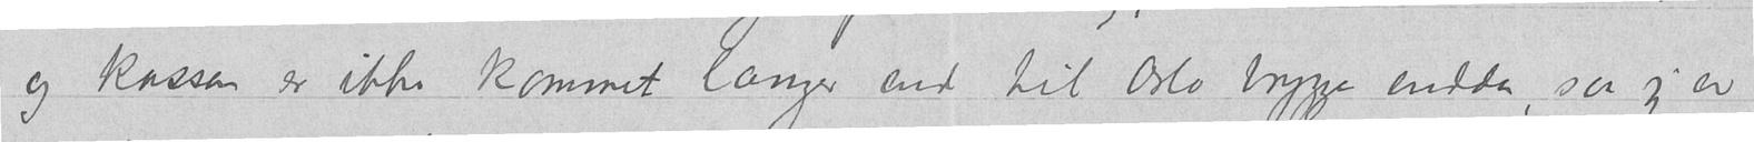og kassen er ikke kommet længer end til Oslo brygge endda, saa jeg er
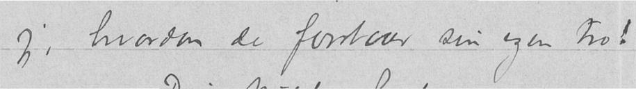jeg, hvordan de forstaar sin egen tro!
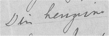Din hengivne
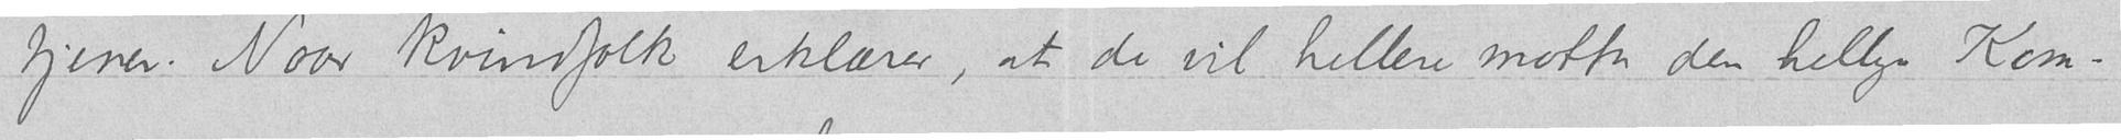tjener. Naar kvindfolk erklærer, at de vil hellere motta den hellige Kom-
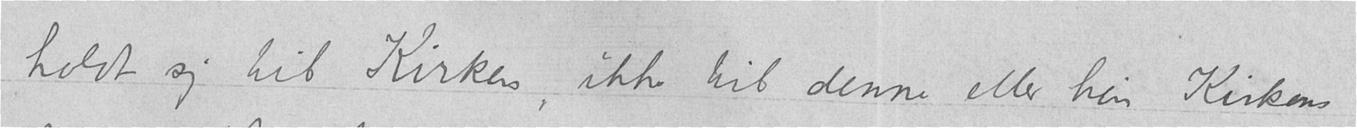holdt sig til Kirken, ikke til denne eller hin Kirkens
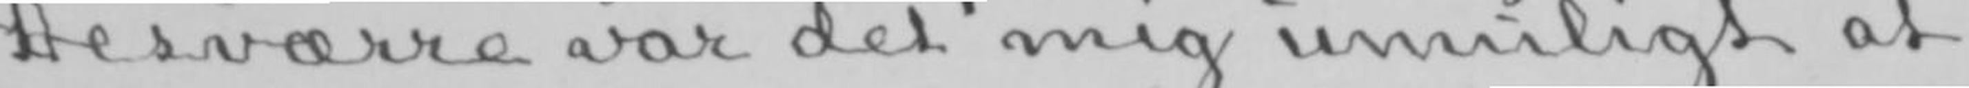Desværre var det mig umuligt at
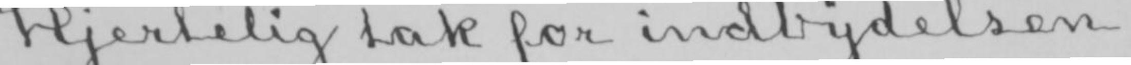Hjertelig tak for indbydelsen!
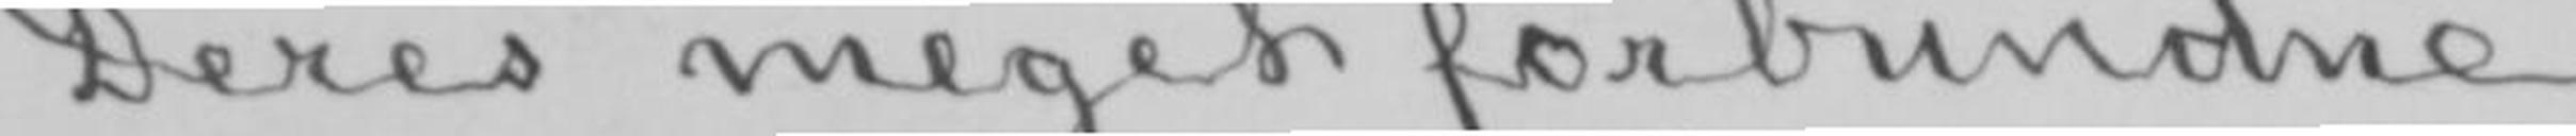Deres meget forbundne
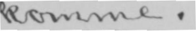komme.
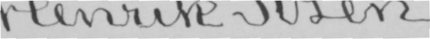Henrik Ibsen.
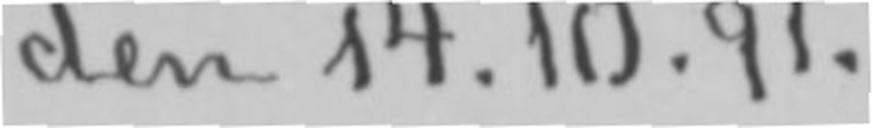den 14.10.91.
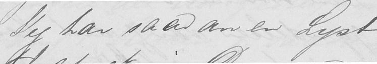Jeg har saadan en Lyst
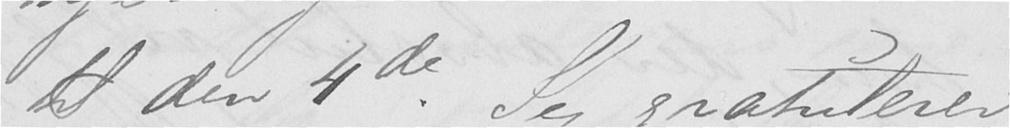til den 4de. Jeg gratulerer
